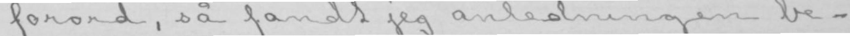forord, så fandt jeg anledningen be-
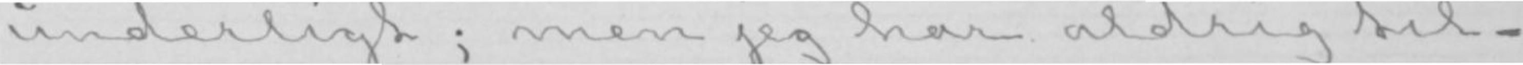underligt; men jeg har aldrig til-
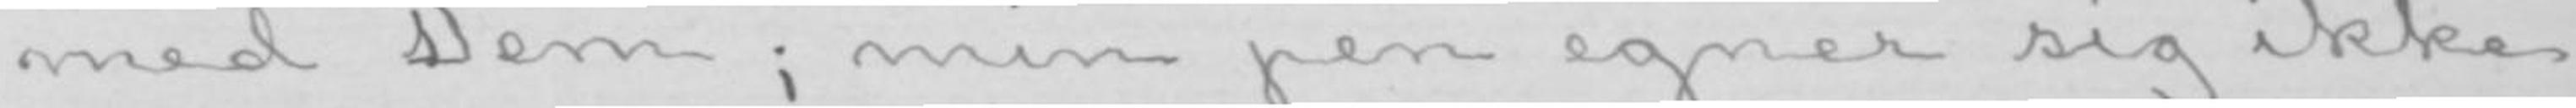med Dem; min pen egner sig ikke
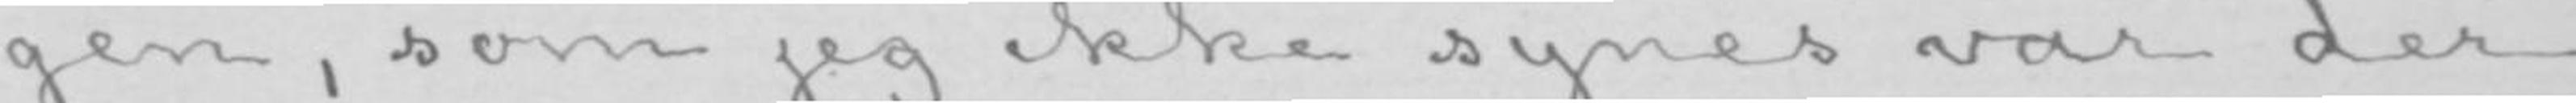gen, som jeg ikke synes var der
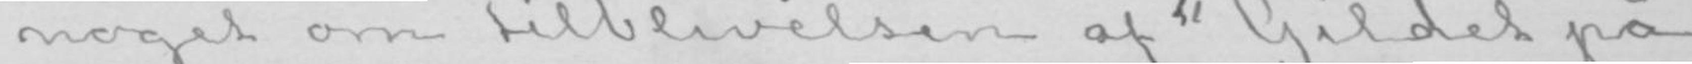noget om tilblivelsen af «Gildet på
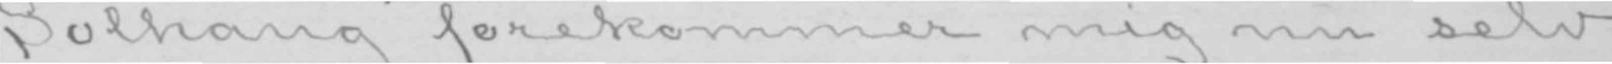Solhaug» forekommer mig nu selv
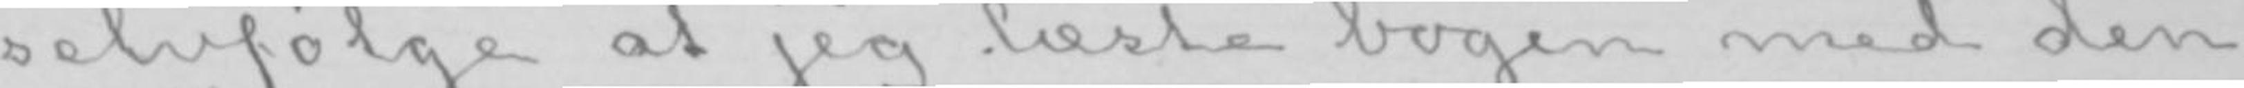selvfølge at jeg læste bogen med den
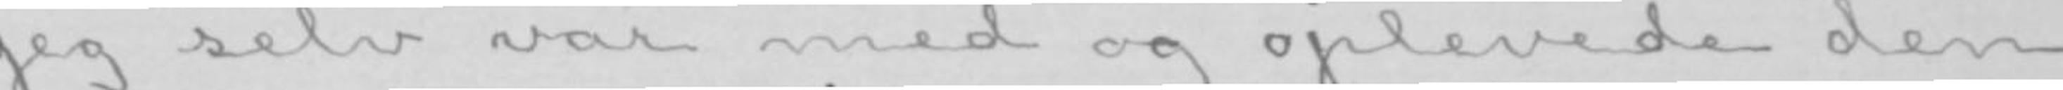jeg selv var med og oplevede den
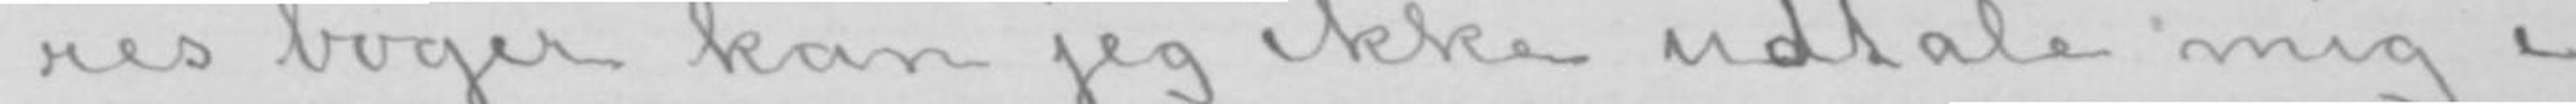res bøger kan jeg ikke udtale mig i
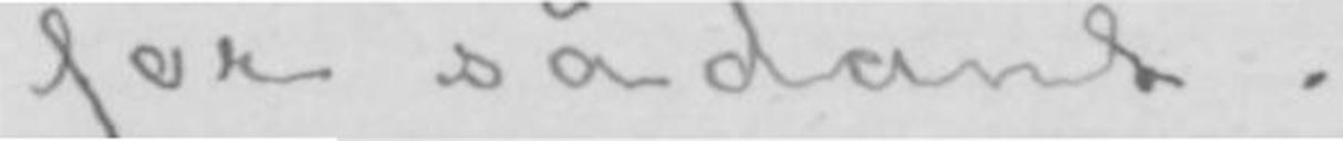for sådant.
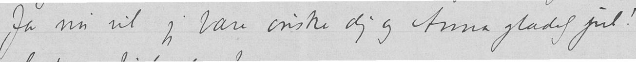Ja nu vil jeg bare ønske dig og Anna glædig jul!
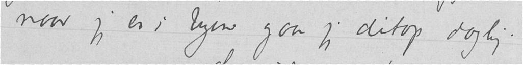naar jeg er i byen gaar jeg ditop daglig.
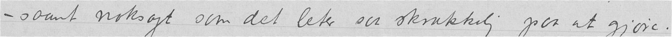– saant noksagt som det leter saa skrækkelig paa at gjøre.
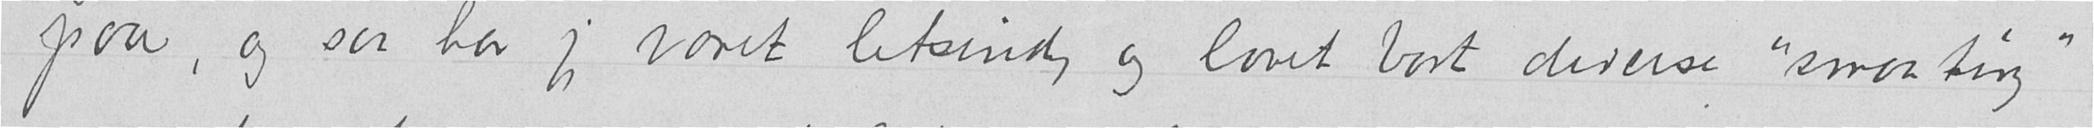paa, og saa har jeg været letsindig og lovet bort diverse "smaating"
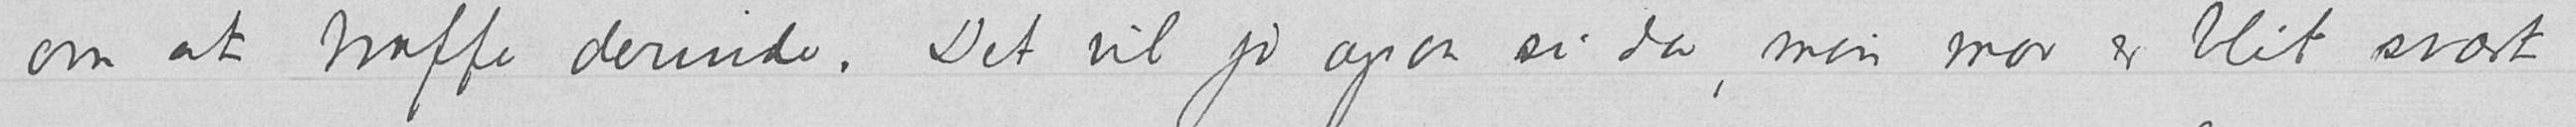om at træffe derinde. Det vil jo ogsaa si da, min mor er blit svært
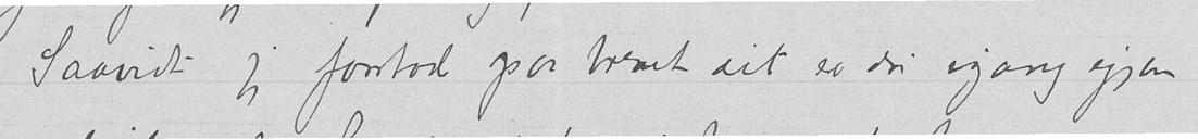Saavidt jeg forstod paa brevet dit er du igang igjen
